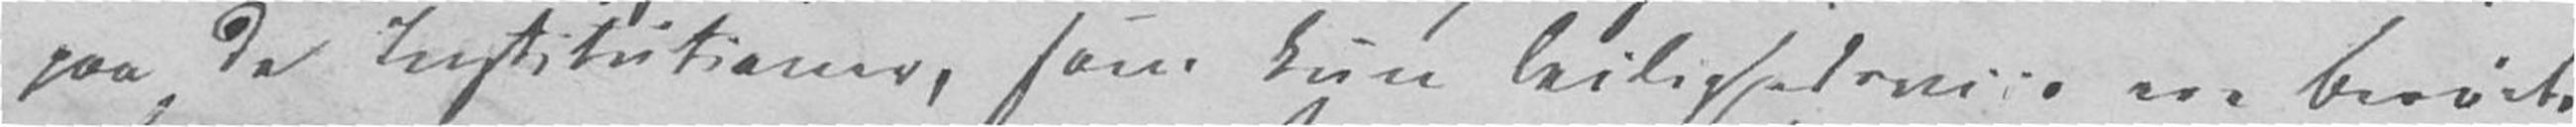paa de Institutioner, som kun leilighedsviis ere berørte
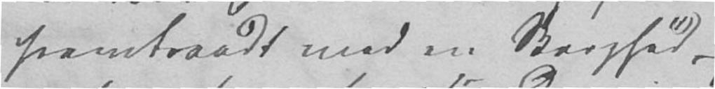fremtraadt med en Skarphed,
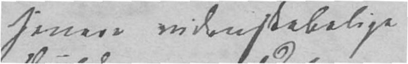senere videnskabelige
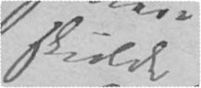skulde
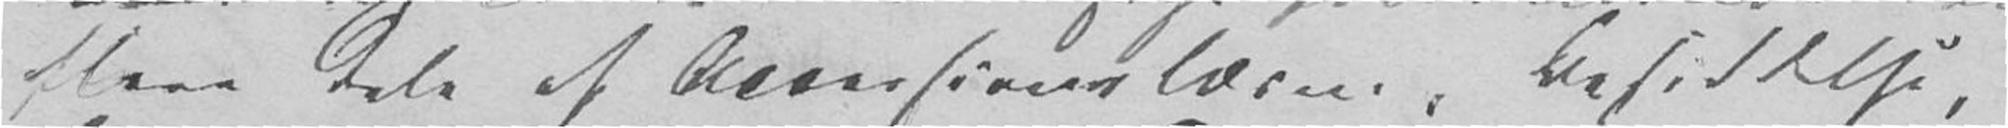flere Dele af Accessionslæren, Besiddelse,
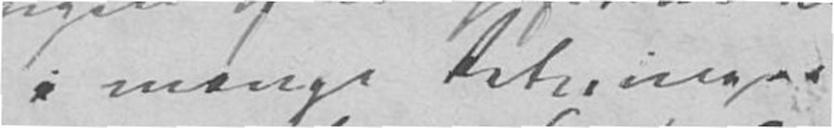i mange Retninger
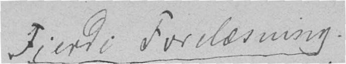Fjerde Forelæsning.
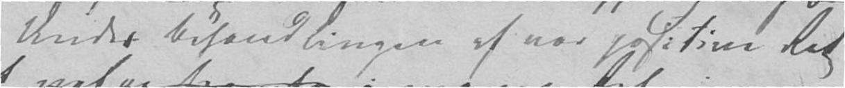Under Behandlingen af vor positive Ret
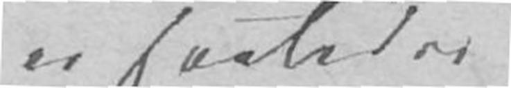er saaledes
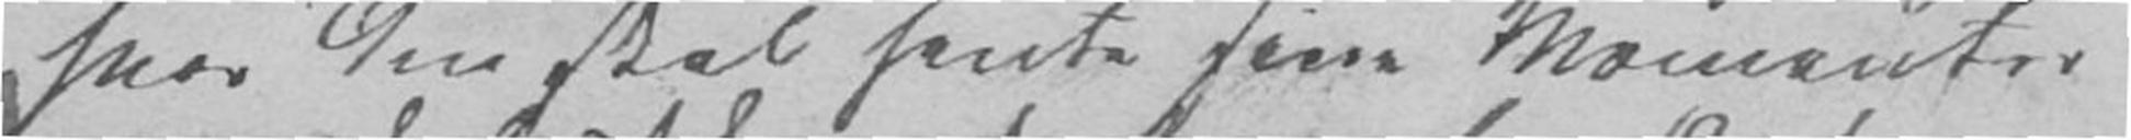hvor den skal hente sine Momenter
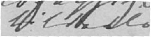tildeels
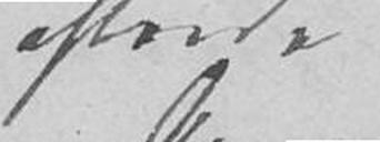ofrede
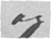og
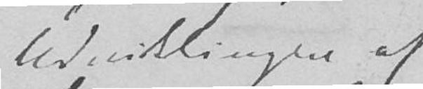Udviklingen af
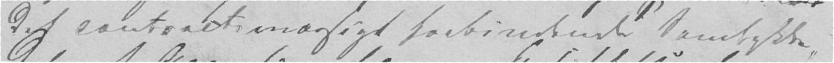det contractsmæssigt forbindende Samtykke,
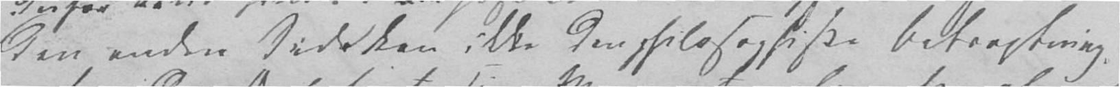den anden Side kan ikke den philosophiske Betragtning,
